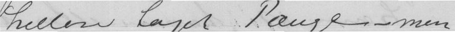hellede taget Pænge - men
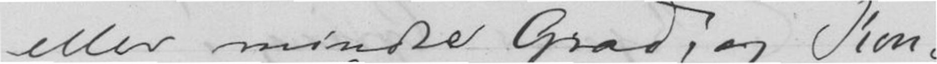eller mindre Grad, og Kon-
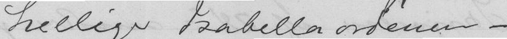hellige Isabellaordenen -
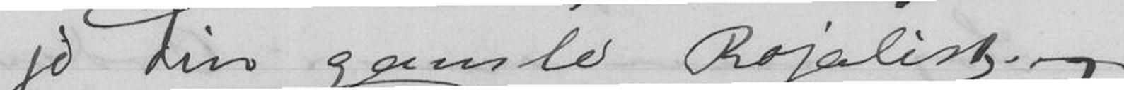jo Din gamle Rojalist og
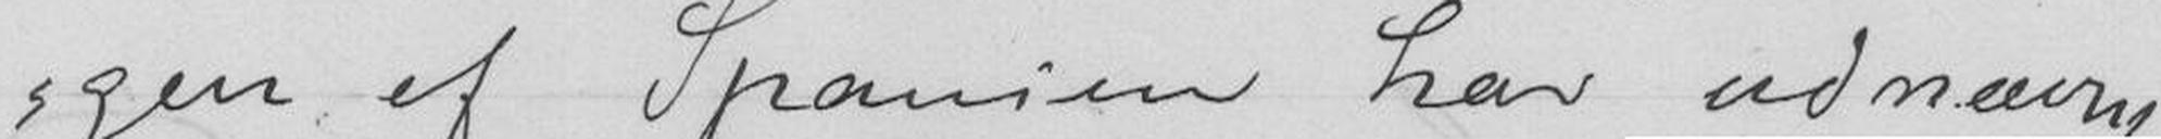gen af Spanien har udnævnt
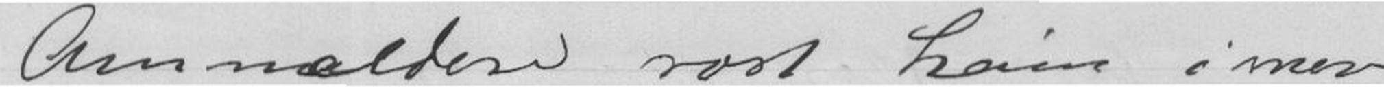Anmældere rost ham i mer
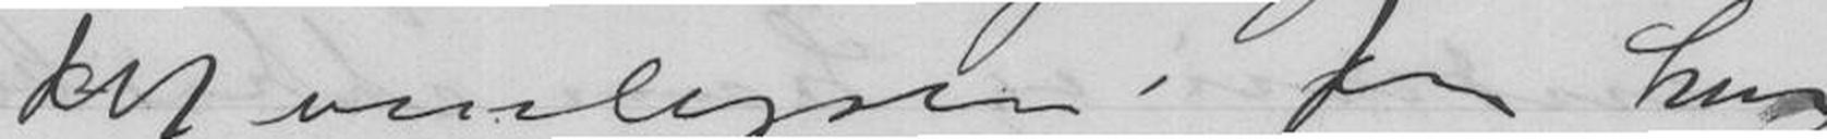det venligste. Din heng
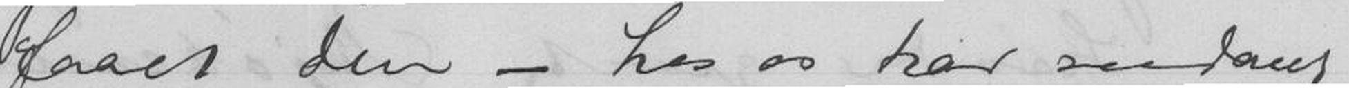faaet den - hos os har saadant
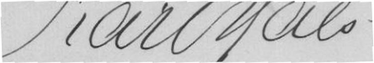Karl Hals
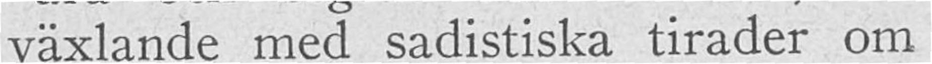växlande med sadistiska tirader om
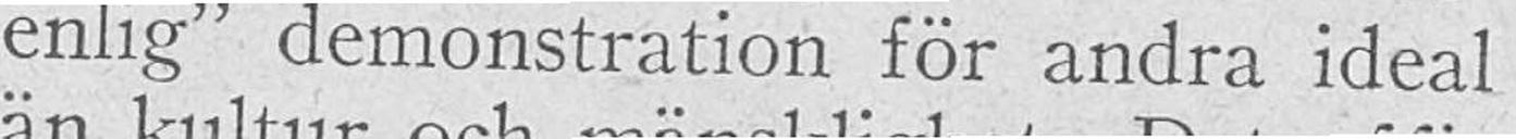enlig" demonstration för andra ideal
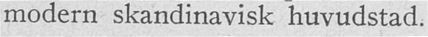modern skandinavisk huvudstad.
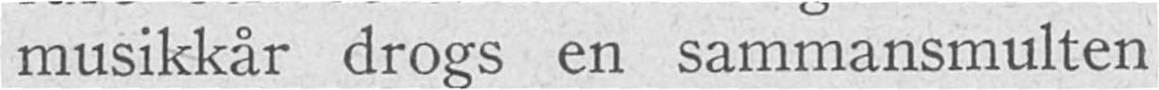musikkår drogs en sammansmulten
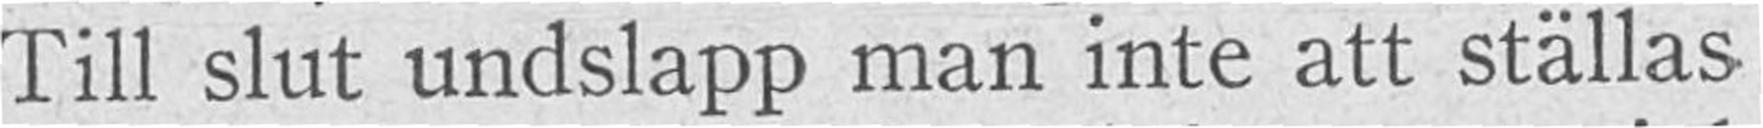Till slut undslapp man inte att ställas
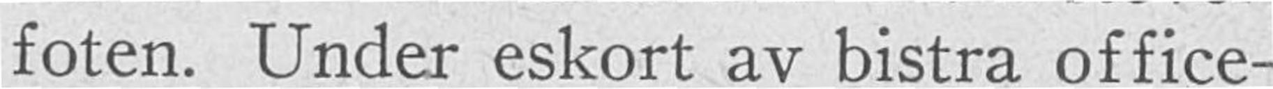foten. Under eskort av bistra office-
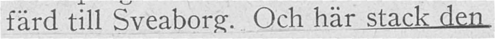färd till Sveaborg. Och här stack den
